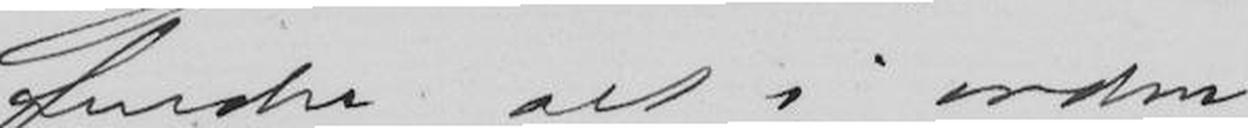finder alt i orden, -
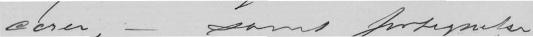cerer, - samt fortegnelse
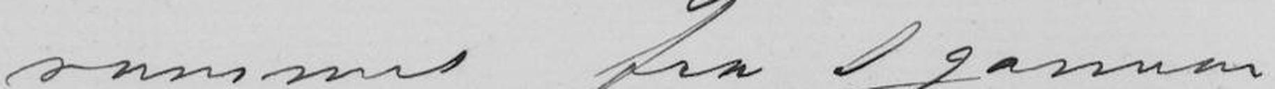rummet fra 1 Januar
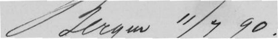Bergen 11/4 90.
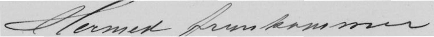Hermed fremkommer
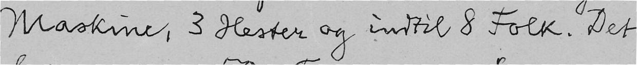Maskine, 3 Hester og indtil 8 Folk. Det
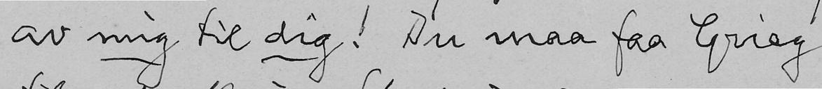av mig til dig! Du maa faa Grieg
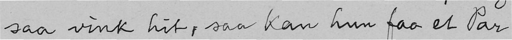saa vink hit, saa kan hun faa et Par
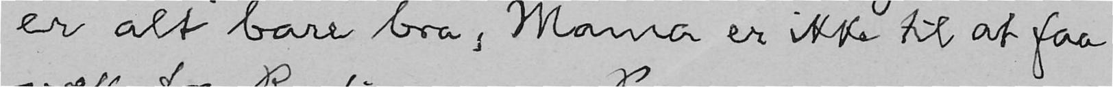er alt bare bra, Mama er ikke til at faa
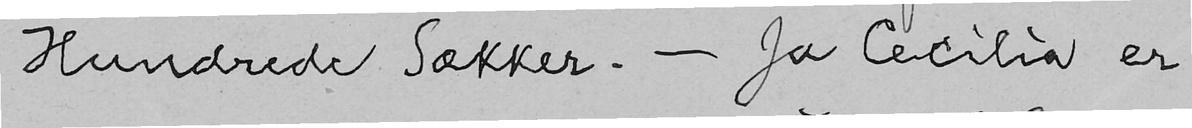Hundrede Sækker. - Ja Cecilia er
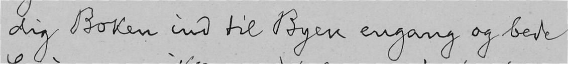dig Boken ind til Byen engang og bede
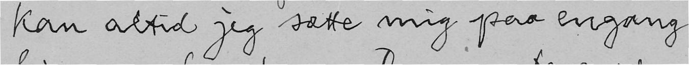kan altid jeg sætte mig paa engang
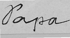Papa
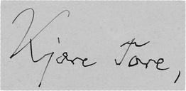Kjære Tore,
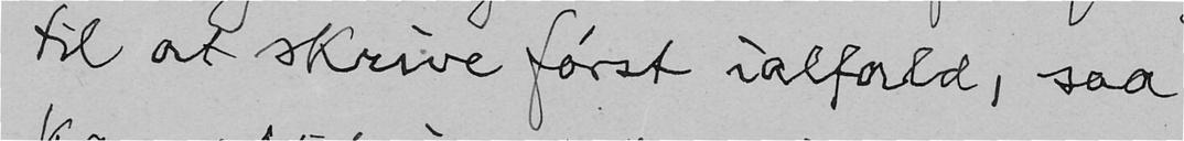til at skrive først ialfald, saa
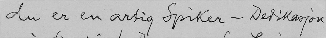du er en artig Spiker - Dedikasjon
